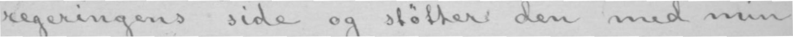regeringens side og stötter den med min
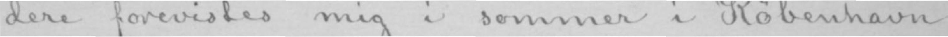dere forevistes mig i sommer i Köbenhavn
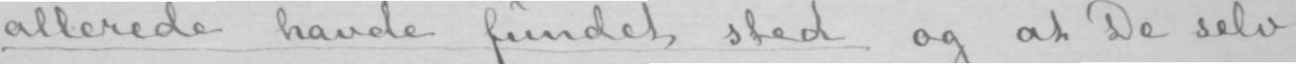allerede havde fundet sted og at De selv
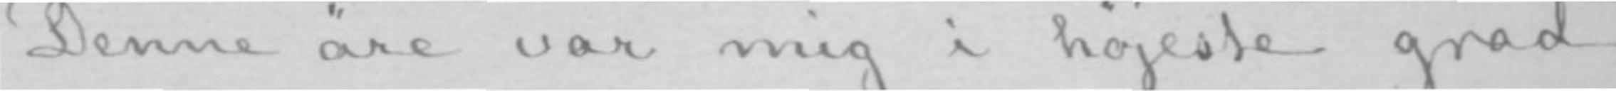Denne äre var mig i höjeste grad
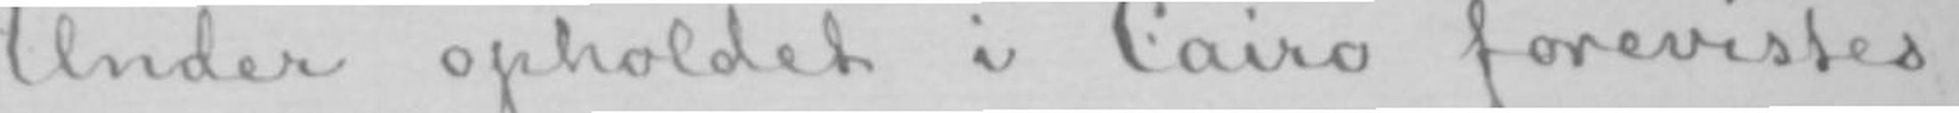Under opholdet i Cairo forevistes
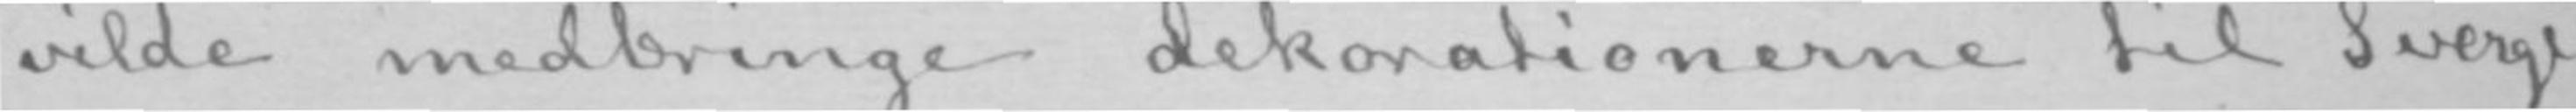vilde medbringe dekorationerne til Sverge.
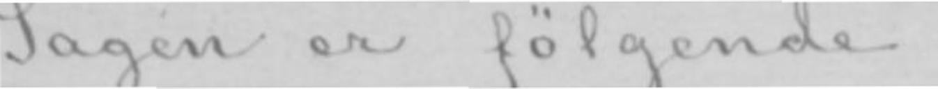Sagen er fölgende:
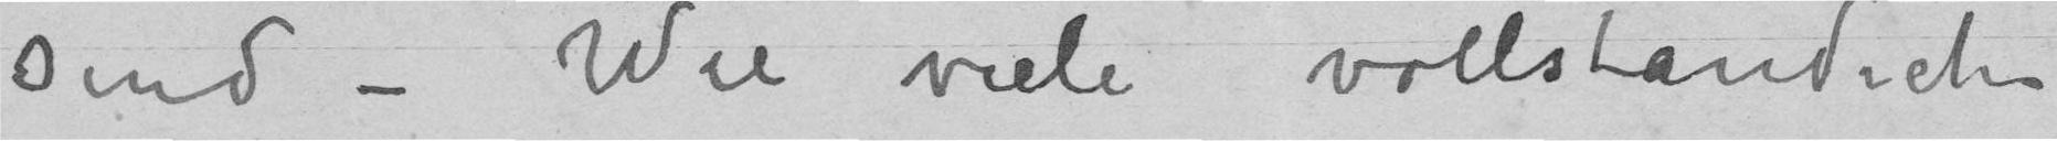sind – Wie viele vollständiche
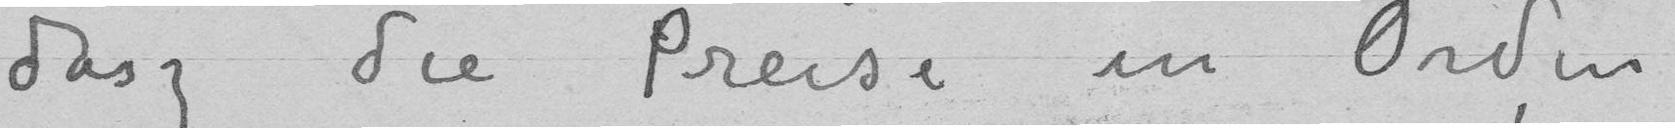dasz die Preise in Orden
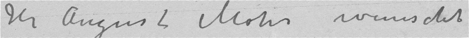Hr August Mohr wunscht
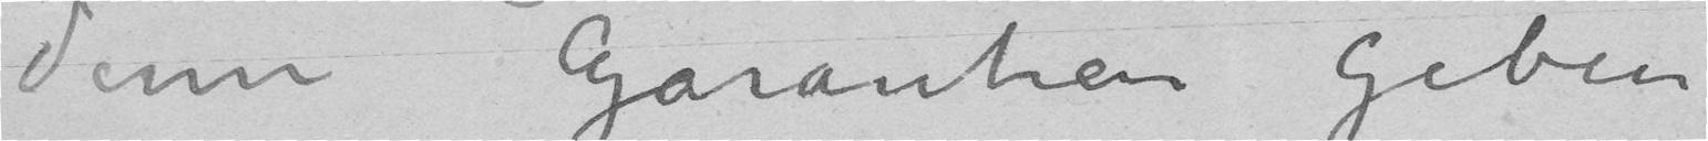dann Garantien geben
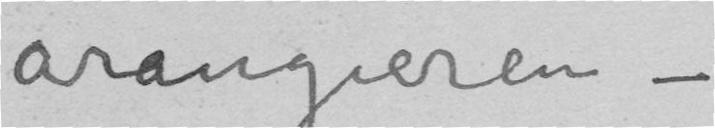arrangieren –
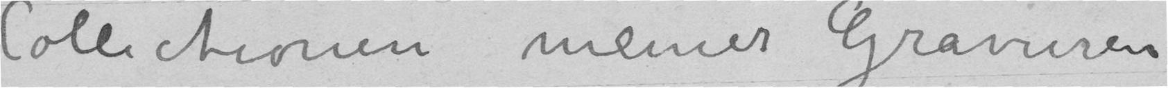Collectionen meiner Gravuren
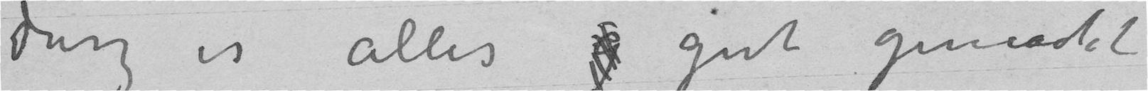dasz es alles g gut gemacht
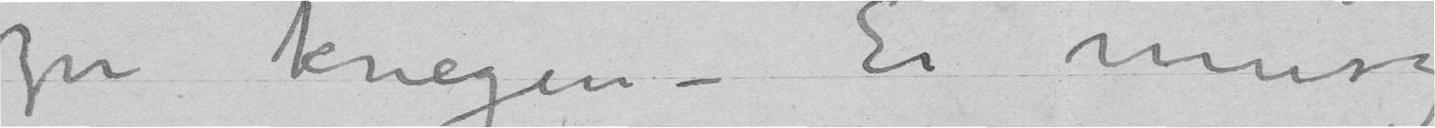zu kriegen – Er musz
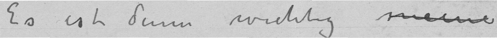Es ist dann wichtig meine
